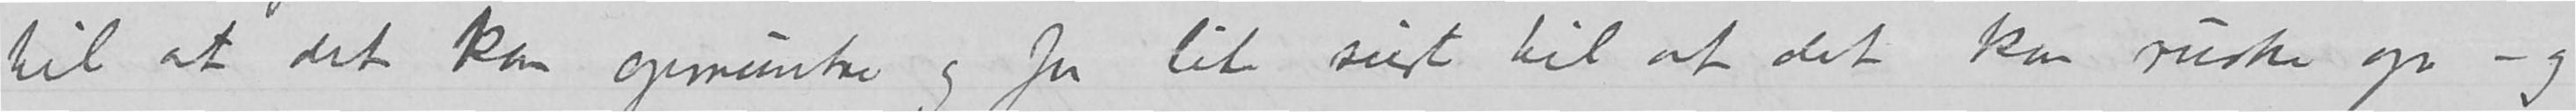til at det kan opmuntre og for lite sint til at det kan ruske op – og
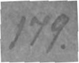179.
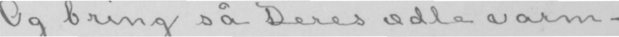Og bring så Deres ædle varm-
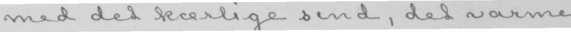med det kærlige sind, det varme
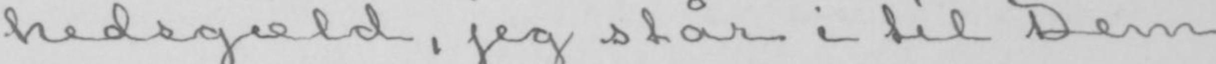hedsgæld, jeg står i til Dem,
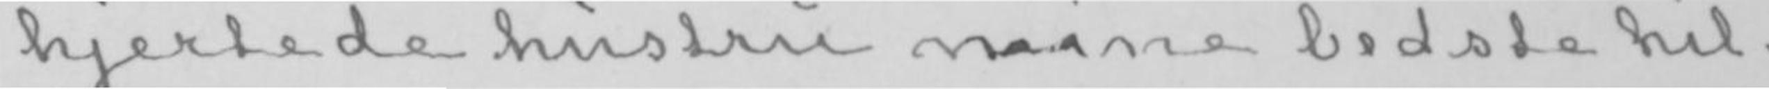hjertede hustru mine bedste hil-
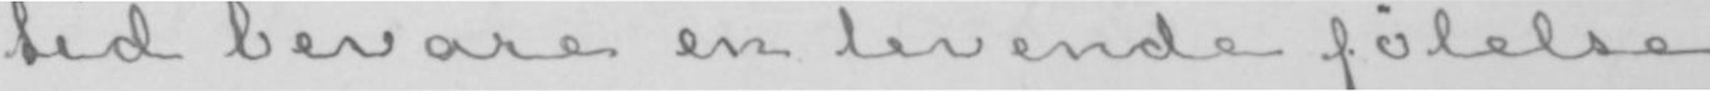tid bevare en levende følelse
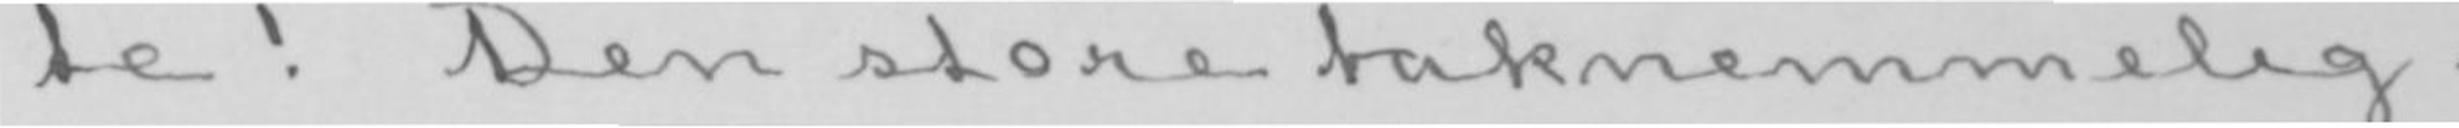te! Den store taknemmelig-
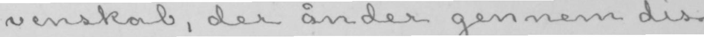venskab, der ånder gennem dis-
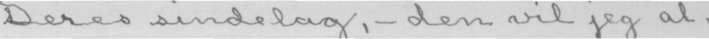Deres sindelag,- den vil jeg al-
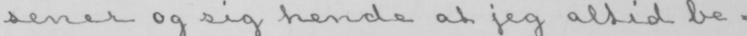sener og sig hende at jeg altid be-
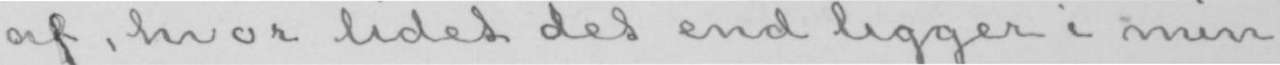af, hvor lidet det end ligger i min
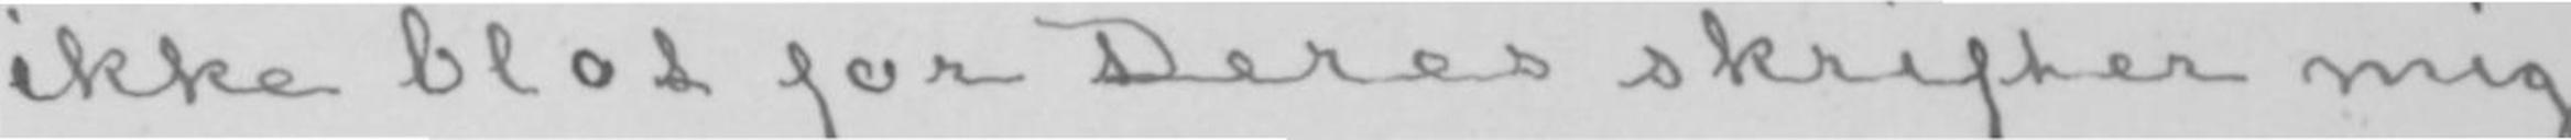ikke blot for Deres skrifter mig
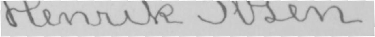Henrik Ibsen.
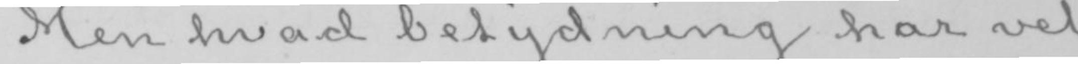Men hvad betydning har vel
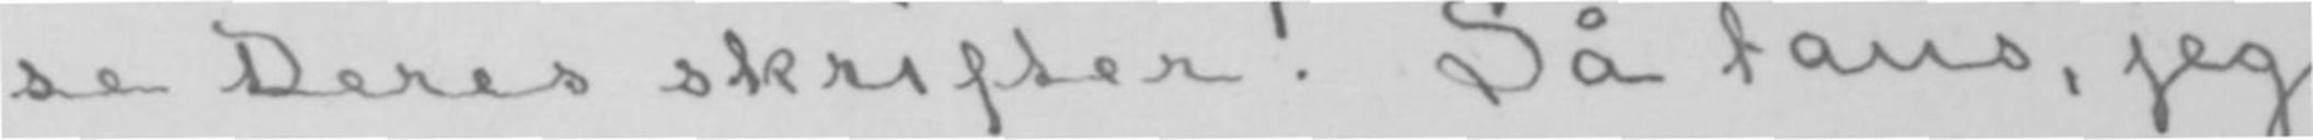se Deres skrifter! Så taus, jeg
